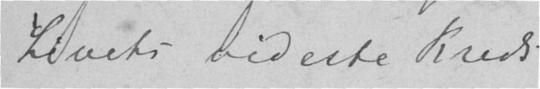Livets videste Kreds
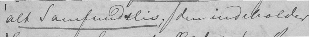alt Samfundsliv; den indeholder
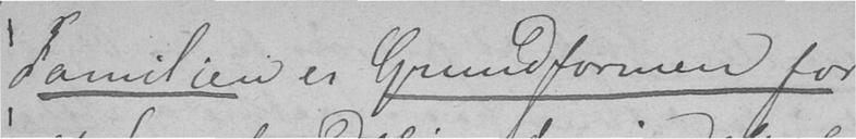Familien er Grundformen for
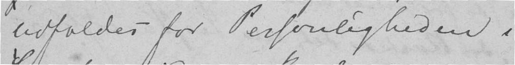udfoldes for Personligheden i
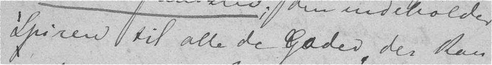Spiren til alle de Goder, der kan
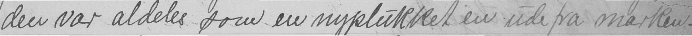den var aldeles som en nyplukket en ude fra marken.
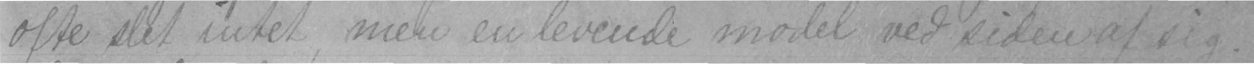ofte slet intet, men en levende model ved siden af sig.
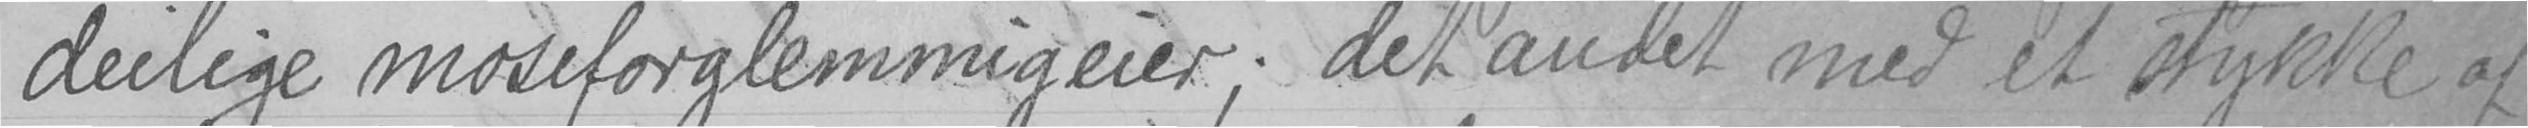deilige moseforglemmigeier; det andet med et stykke af
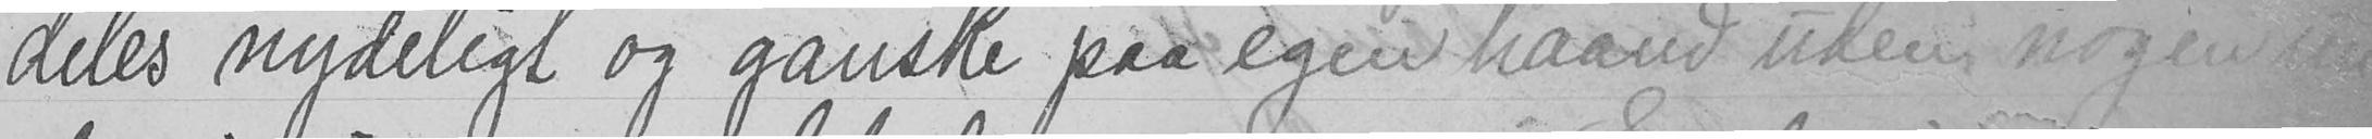deles nydeligt og ganske paa egen haand uden nogen un-
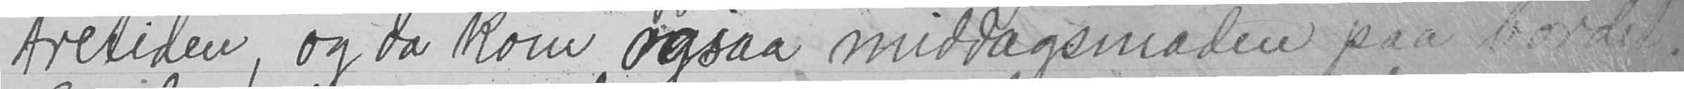tretiden, og da kom ogsaa middagsmaden paa bordet.
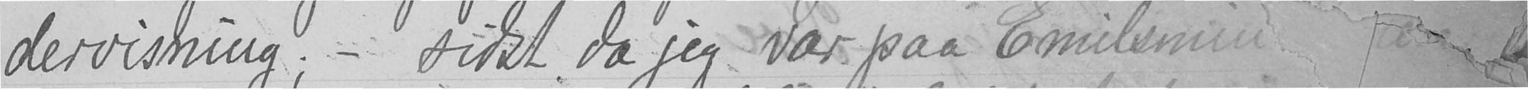dervisning; - sidst, da jeg var paa Emilsminde
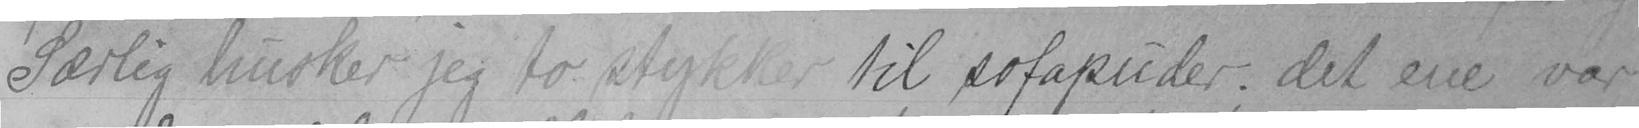Særlig husker jeg to stykker til sofapuder; det ene var
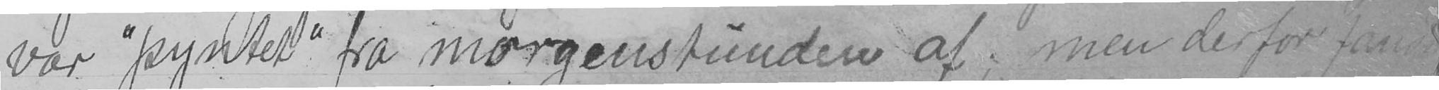var "pyntet" fra morgenstunden af; men derfor fandt
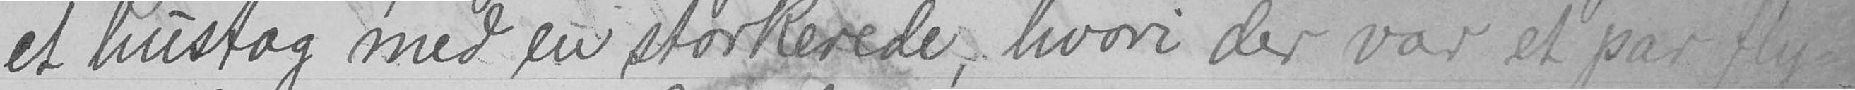et hustag med en storkerede, hvori der var et par fly-
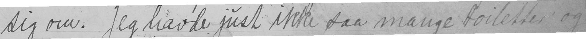sig om. Jeg havde just ikke saa mange toiletter og
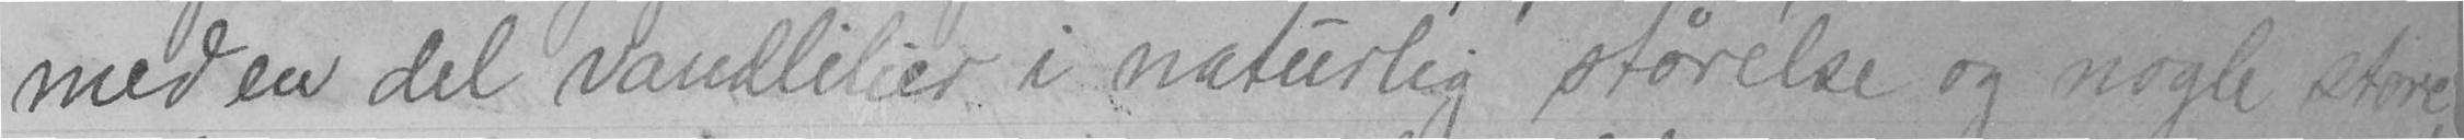med en del vandlilier i naturlig størelse og nogle store,
